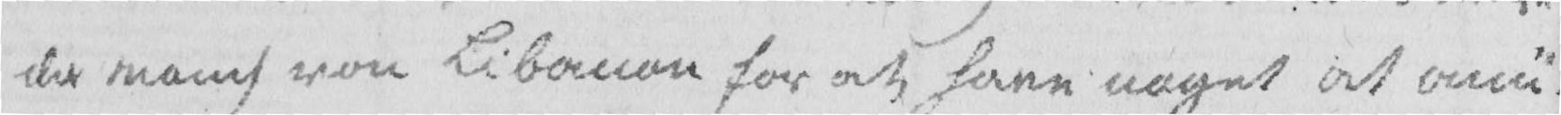der monch von Libanon for at have noget at amu-
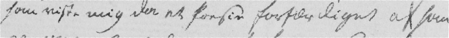som viste mig der et Poesie forfærdiget af ham
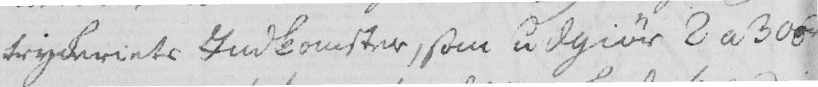tryckeriets Indkomster, som udgiør 2 a 300 rd
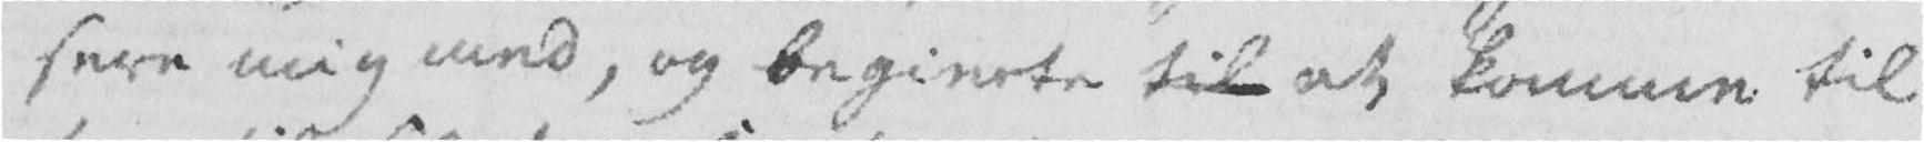sere mig med, og begierte til at komme til
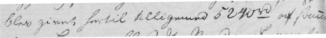blev givet hertil tilligemed 5240 rd af samme.
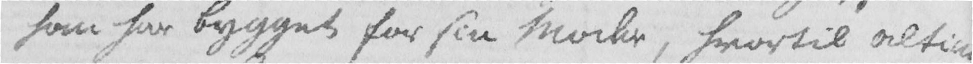han har bygget for sin Moder, hvortil alting
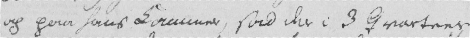og paa hans Kammer, sad der i 3 Qvarteer
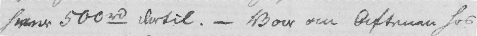hver 500 rd dertil. Var om Aftenen hos
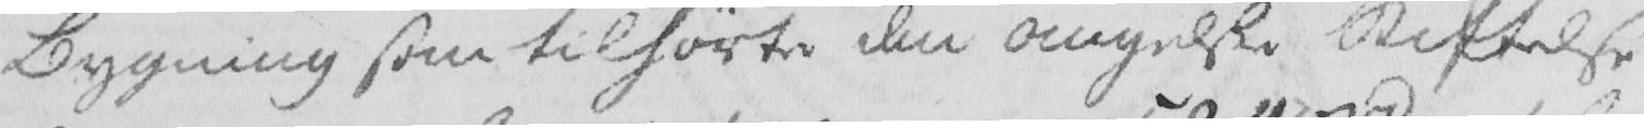Bygning som tilhørte den angelske Stiftelse
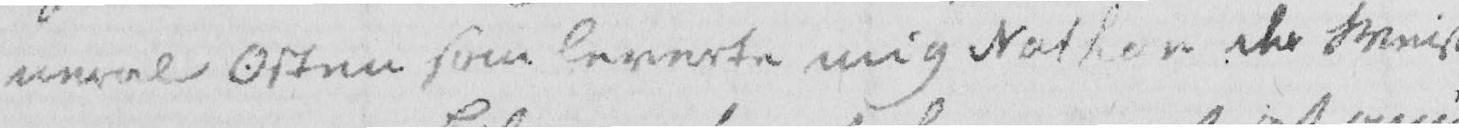neral Osten som leverte mig Nathan der Weise
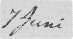7 Juni
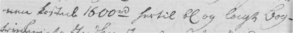nen kostede 1600 rd hertil bl og lagt Bog-
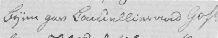byen gav Cancelliraad Gost
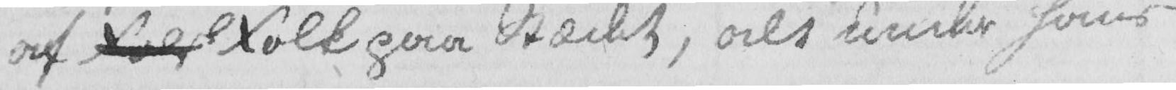af  Folk paa Stædet, alt under hans
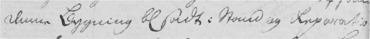Denne Bygning bl sadt i stand og Reparatio-
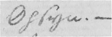Opsyn.
